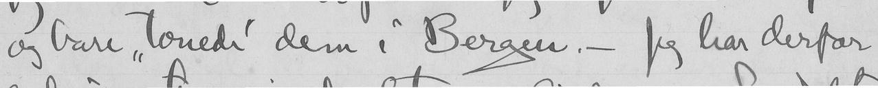og bare "tonede" dem i Bergen. - Jeg har derfor
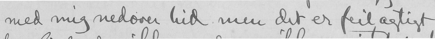med mig nedover hid men det er feilagtigt
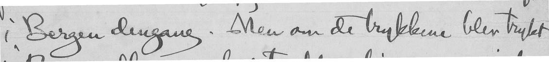i Bergen dengang. Men om de trykkene blev trykt
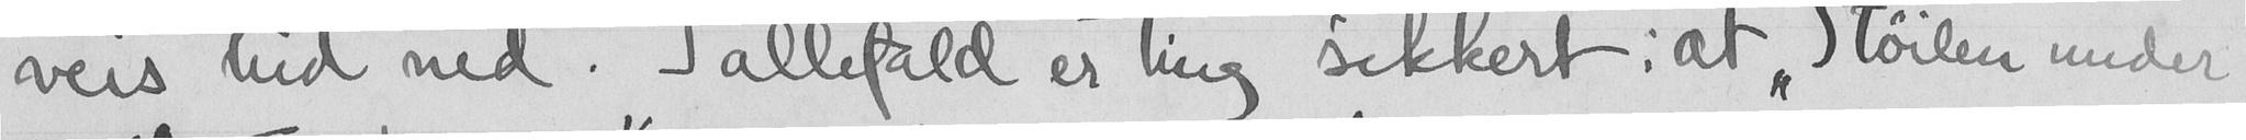veis hid ned. I allefald er ting sikkert : at "Støilen under
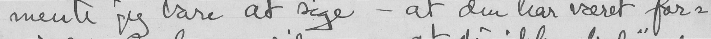mente jeg bare at sige - at den har været "for-
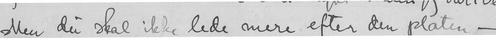Men du skal ikke lede mere efter den platen -
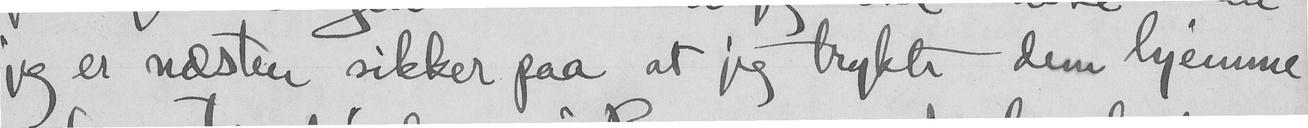jeg er næsten sikker paa at jeg trykte dem hjemme
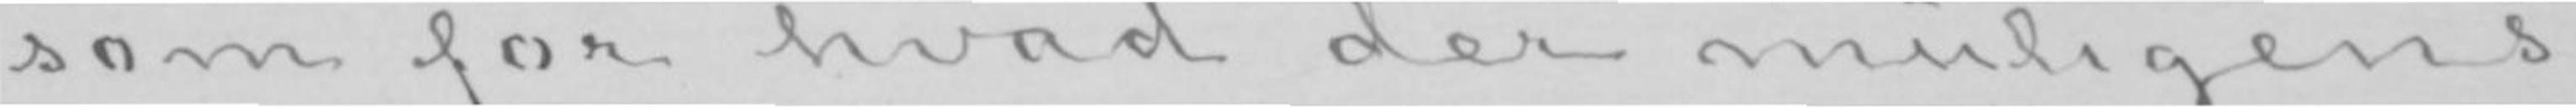som for hvad der muligens
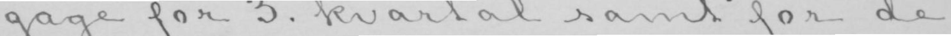gage for 3. kvartal samt for de
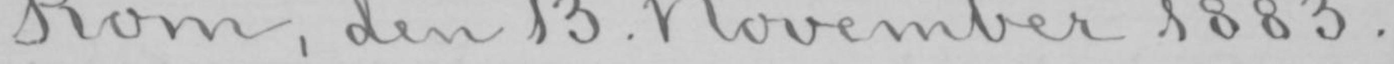Rom, den 13. November 1883.
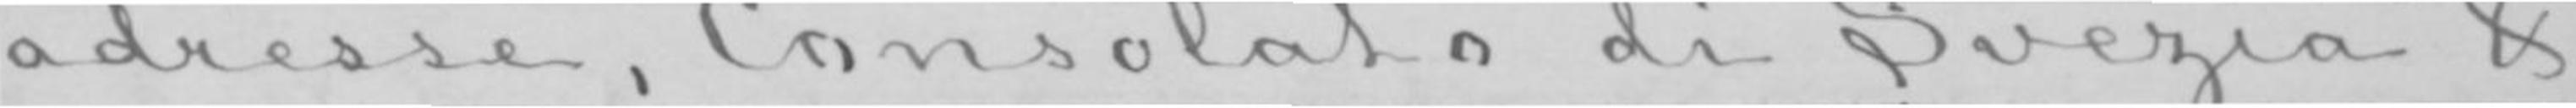adresse, Consolato di Svezia &
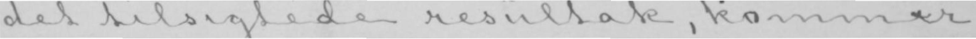det tilsigtede resultak, kommer
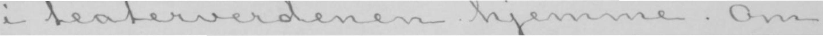i teaterverdenen hjemme. Om
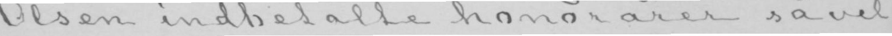Olsen indbetalte honorarer såvel
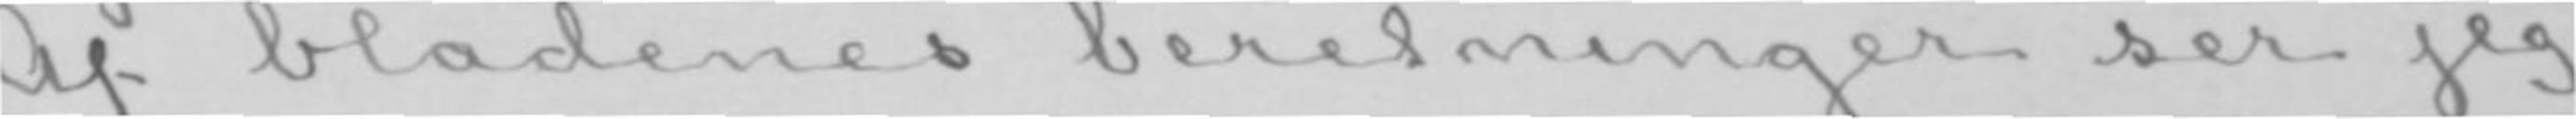Af bladenes beretninger ser jeg
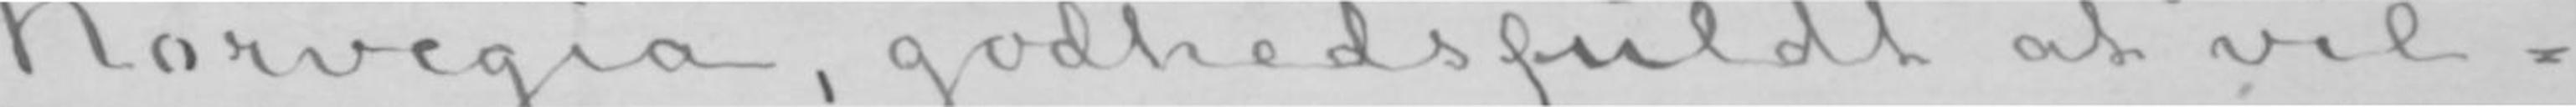Norvegia, godhedsfuldt at vil-
